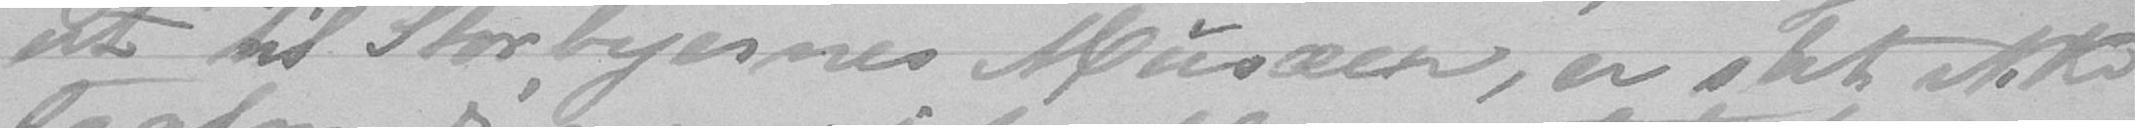ut til Storbyernes Musæer, er slet ikke
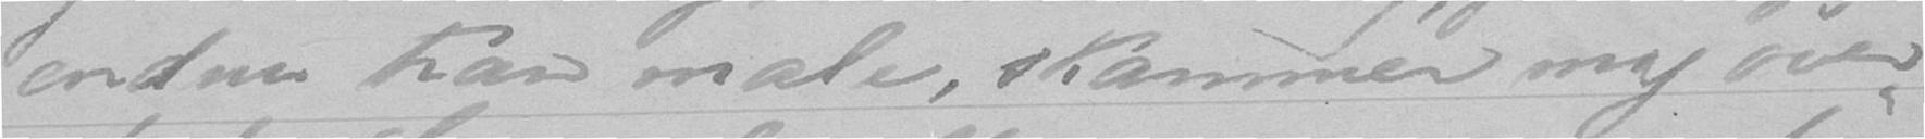endnu kan male, skammer mig over
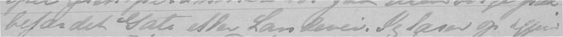befærdet Gate eller Landevei. Jeg læser op igjen
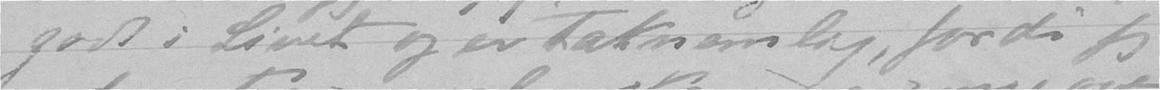godt i Livet og er Taknemlig, fordi jeg
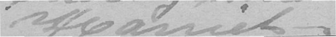Harriet.
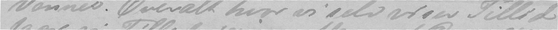Venner. Overalt hvor vi selv viser Tillid
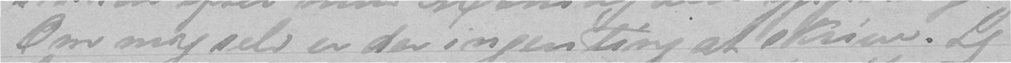Om mig selv er der ingenting at skrive. Jeg
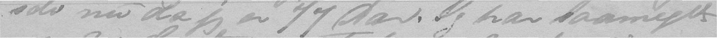selv nu da jeg er 77 Aar. Jeg har saameget
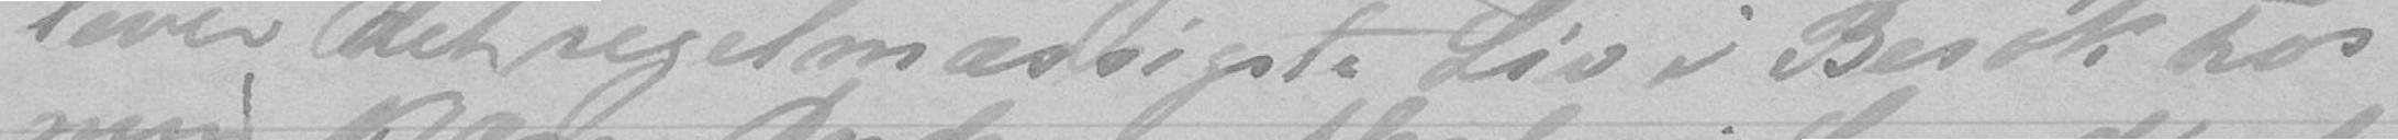lever det regelmæssigste Liv i Besøk hos
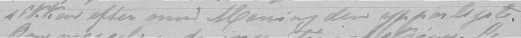sikken efter min Mening den ypperligste.
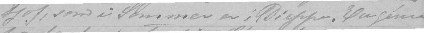tjof, som i Sommer er i Dieppe. Eugénie
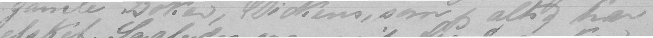gamle Bøker, Dickens, som jeg altid har
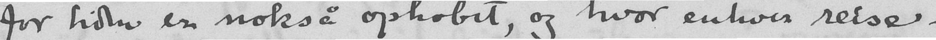for tiden er nokså ophobet, og hvor enhver reise-
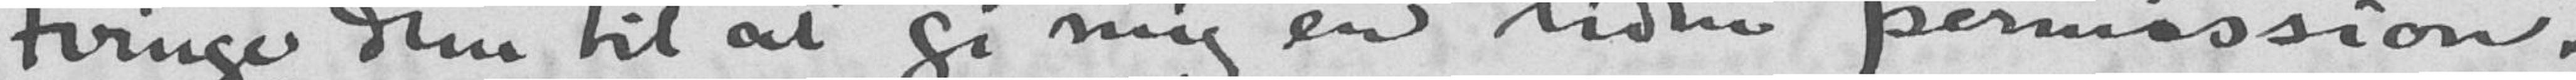tvinge dem til at gi mig en liden permission.
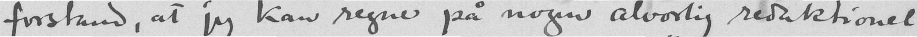forstand, at jeg kan regne på nogen alvorlig redaktionel
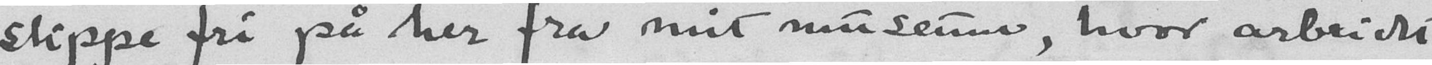slippe fri på her fra mit museum, hvor arbeidet
